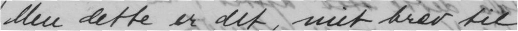Men dette er det, mit brev til
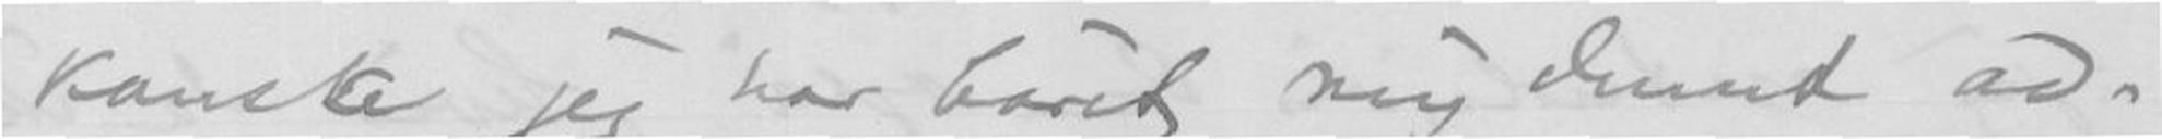kanske jeg har båret mig dumt ad.
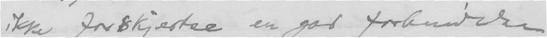ikke forskjertse en god forbindelse
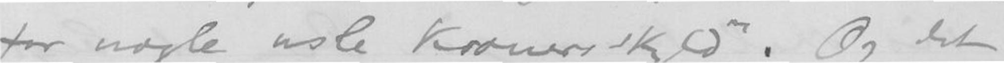for nogle usle Kroner skyld". Og det
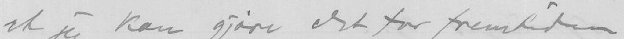at jeg kan gjøre det for fremtiden
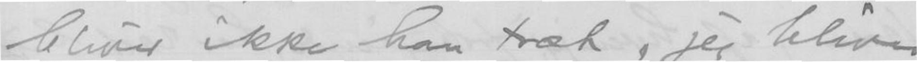bliver ikke han træt, jeg bliver
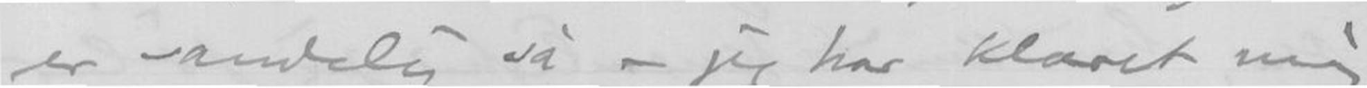er sandelig så - jeg har klaret mig
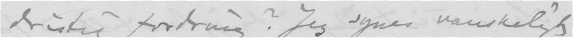dristig fordring? Jeg synes vanskeligt
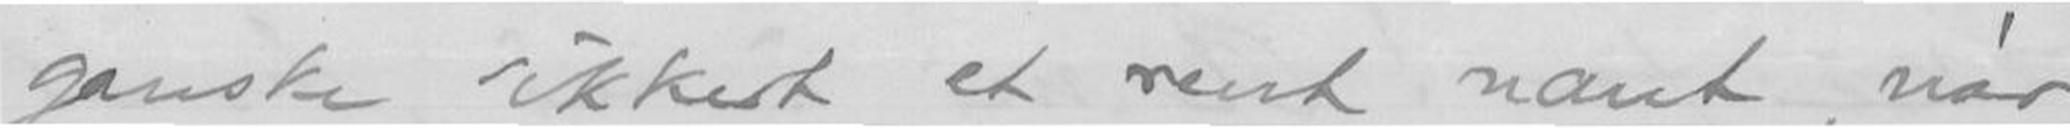ganske sikkert et rent naut, når
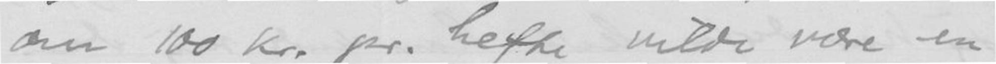om 100 kr. pr. hefte vilde være en
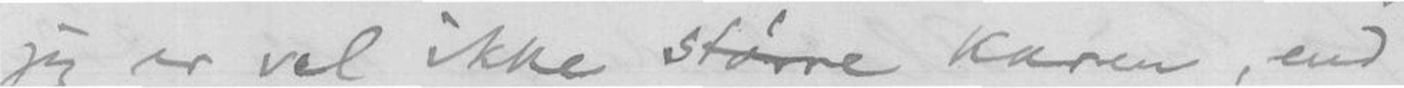jeg er vel ikke større karen, end
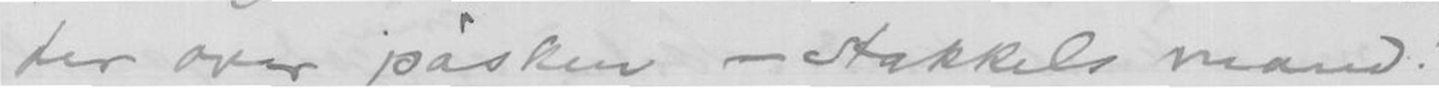ter over påsken - stakkels mand!
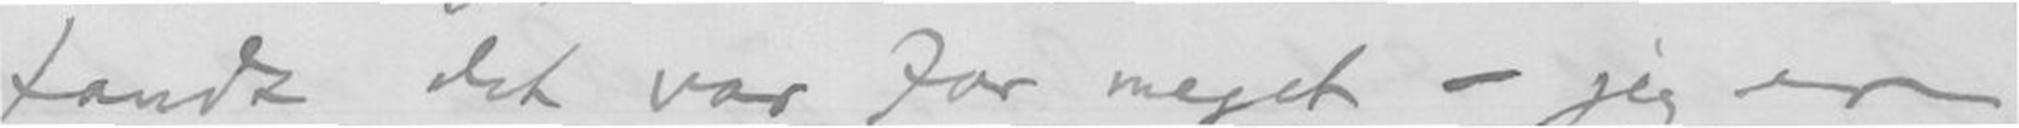fandt det var for meget - jeg er
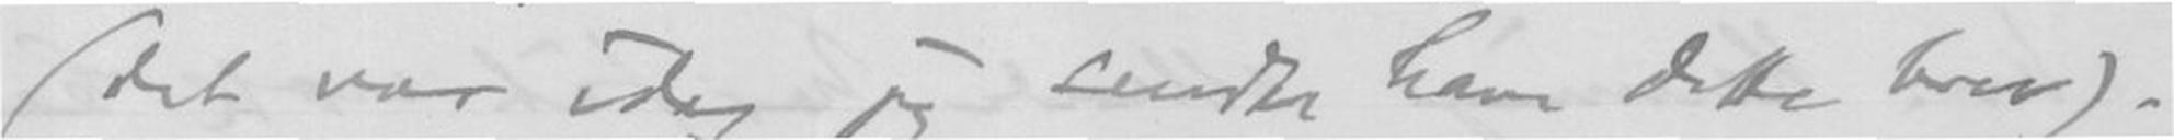(det var idag jeg sendte ham dette brev).
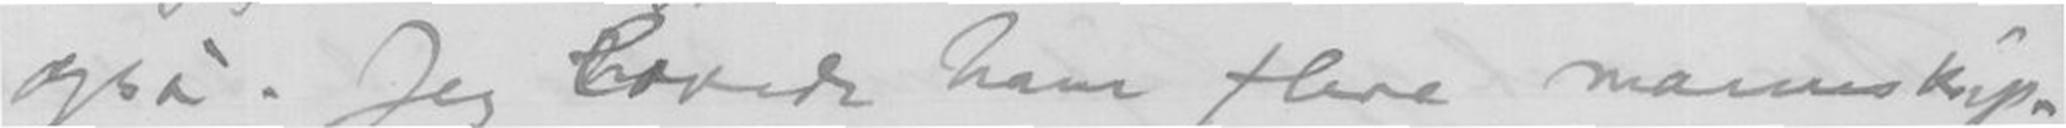også. Jeg lovede ham flere manuskrip-
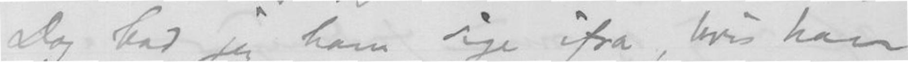Dog bad jeg ham sige ifra, hvis han
